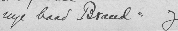nye baad "Brand" og
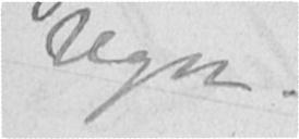regn.
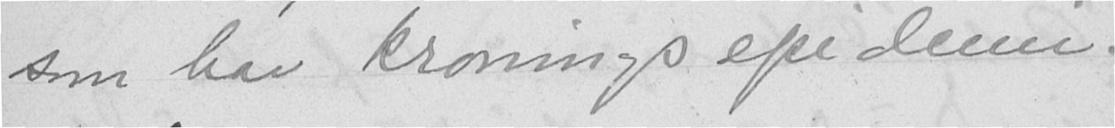som har kroningsepidemi.
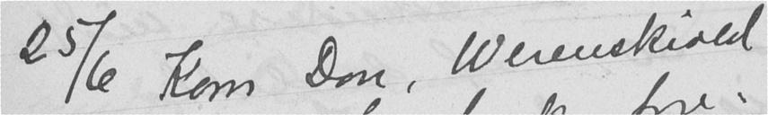25/6 Kom Don, Werenskiold
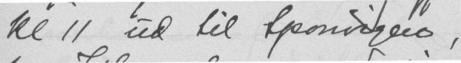kl 11 ud til Sponvigen,
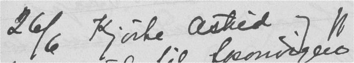26/6 Kjørte Astrid og jeg
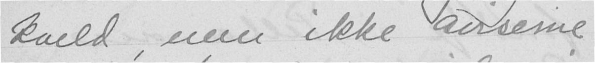kveld, men ikke aviserne
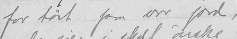for tørt paa vor jord,
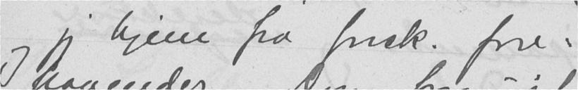og jeg hjem fra forsk. fore-
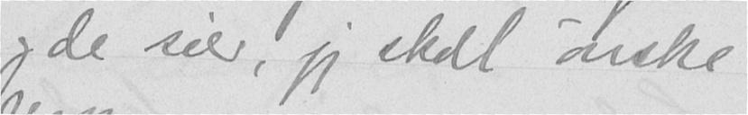og de sier, jeg skal ønske
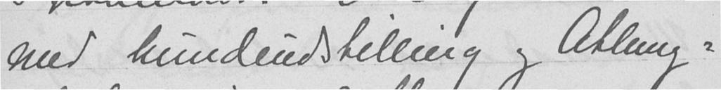med hundeudstilling og Atlung-
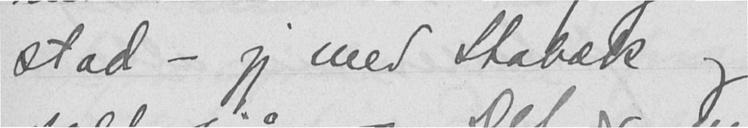stad - jeg med Stabæk og
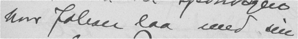hvor Johan laa med sin
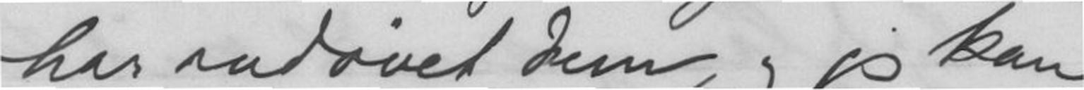har indøvet dem, jeg kan
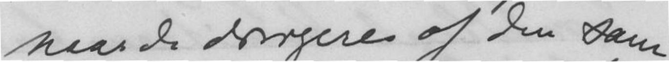naar de dirigeres af den som
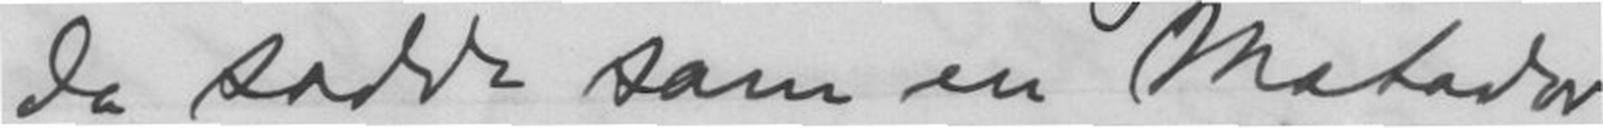da sidde som en Matador
